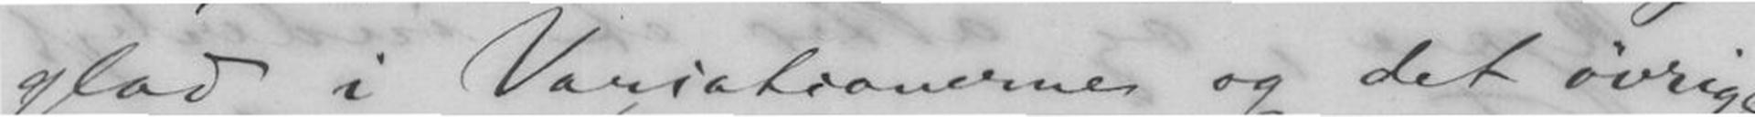glad i Variationerne og det øvrige
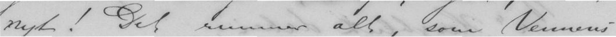nyt! Det rummer alt, som Vennens
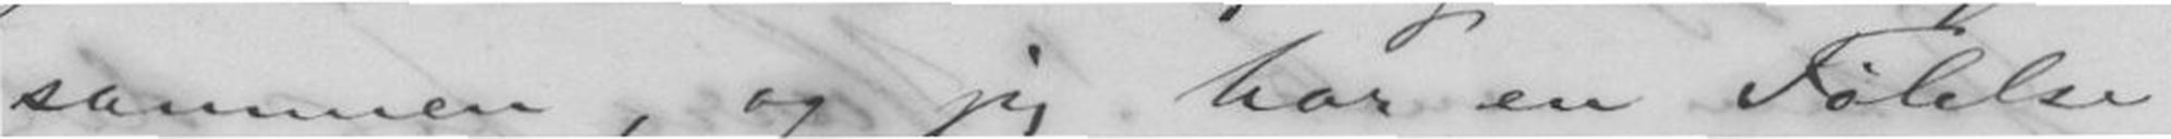sammen, og jeg har en Følelse
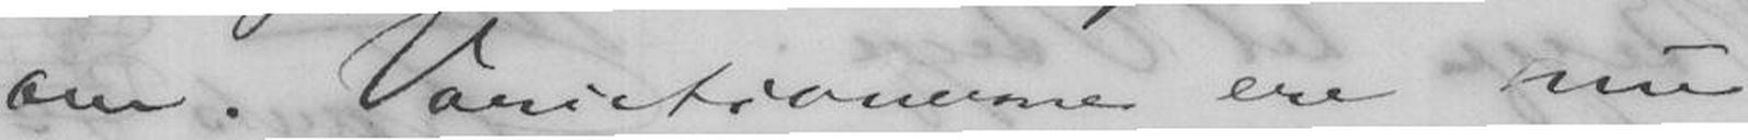om. Varietionerne ere nu
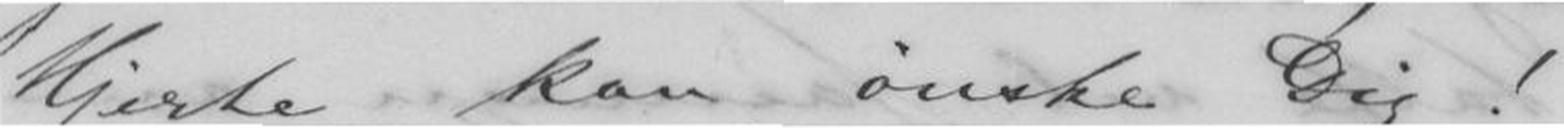Hjerte kan ønske Dig!
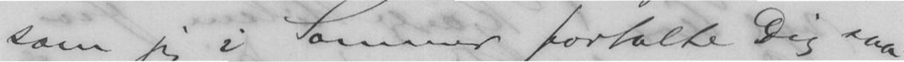som jeg i Sommer fortalte Dig saa
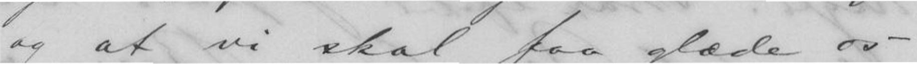og at vi skal faa glæde os
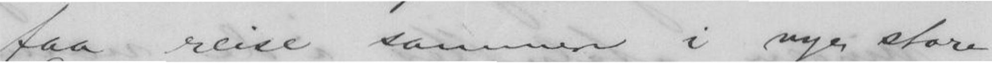faa reise sammen i nye store
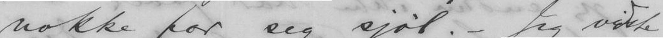nokke for seg sjøl. - Jeg vidste
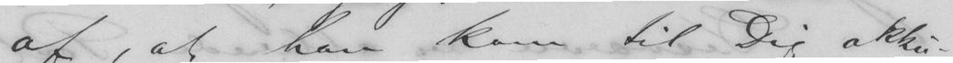af, at han kom til Dig akku-
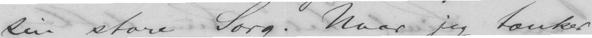sin store Sorg. Naar jeg tænker
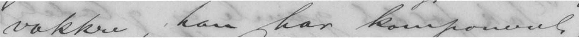vakkre, han har komponeret,
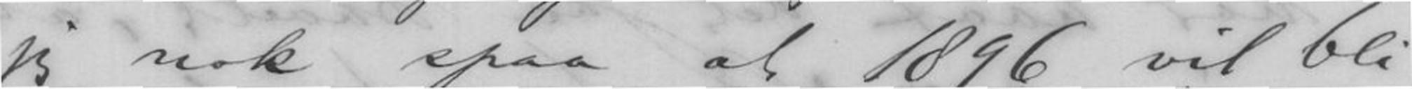jeg nok spaa at 1896 vil bli
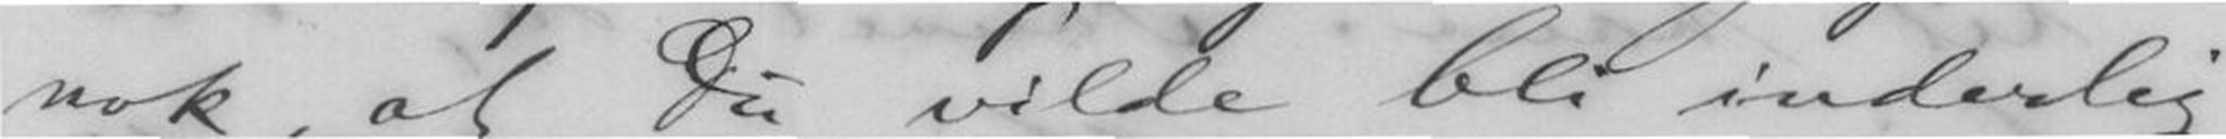nok, at Du vilde bli inderlig
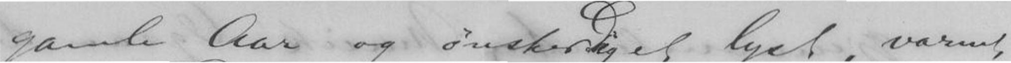gamle Aar og ønsker Dig et lyst, varmt
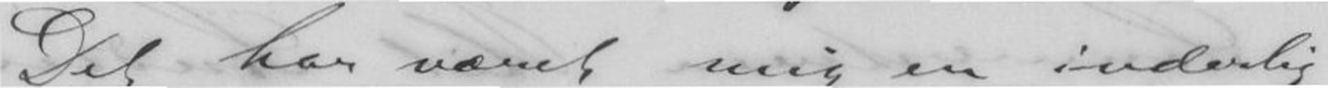Det har været mig en inderlig
